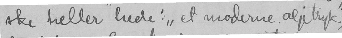ske heller hede: "et moderne oljetryk",
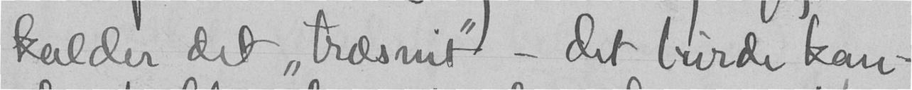kulder det "træsnit" - det burde kan-
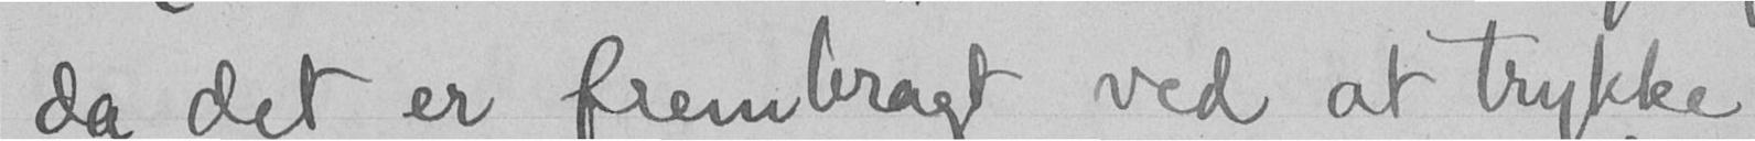da det er frembragt ved at trykke
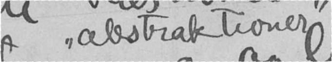"abstraktioner"
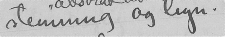stemning og lign. - ikke ved "illederen"
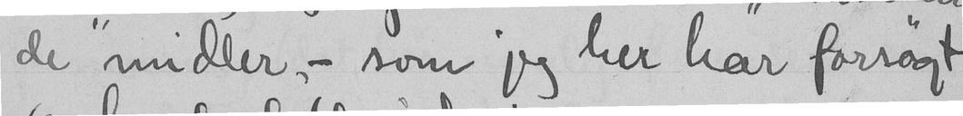de" midler, - som jeg her har forsøgt
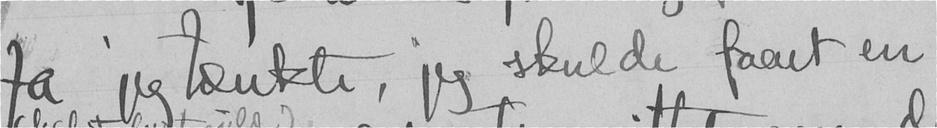Ja jeg tænkte, jeg skulde faaet en
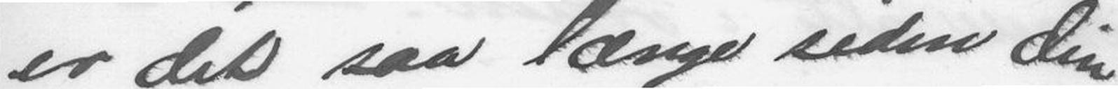er det saa længe siden din
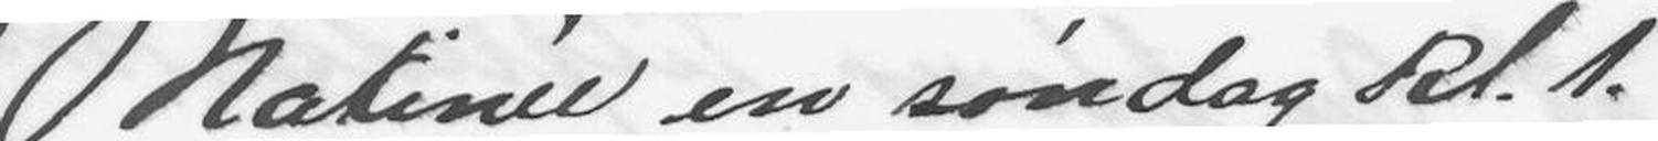Matinèe en søndag kl 1.
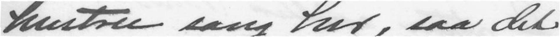hustru sang her, saa det
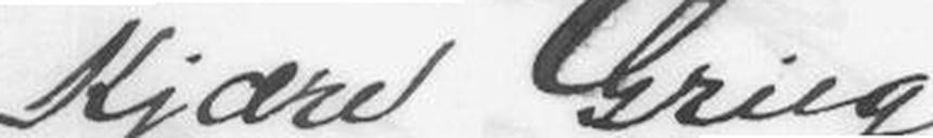Kjære Grieg
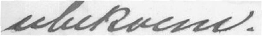ubekvem.
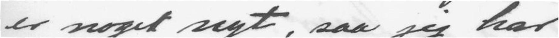er noget nyt, saa jeg har
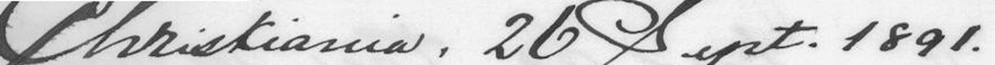Christiania, 26 Sept. 1891.
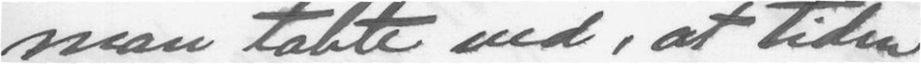man tabte ved, at Tiden
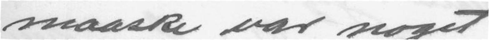maaske var noget
